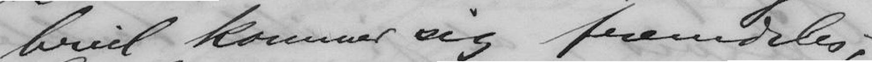briel kommer sig fremdeles,
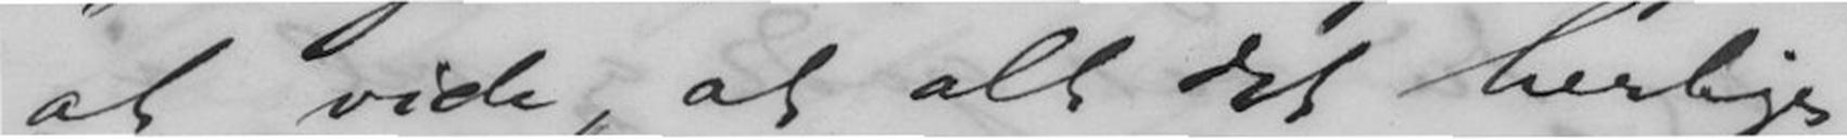at vide, at alt det herlige
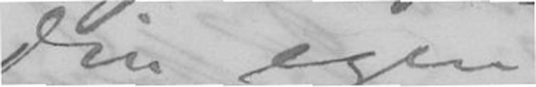Din egen
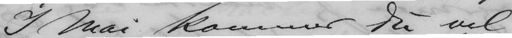I Mai kommer Du vel
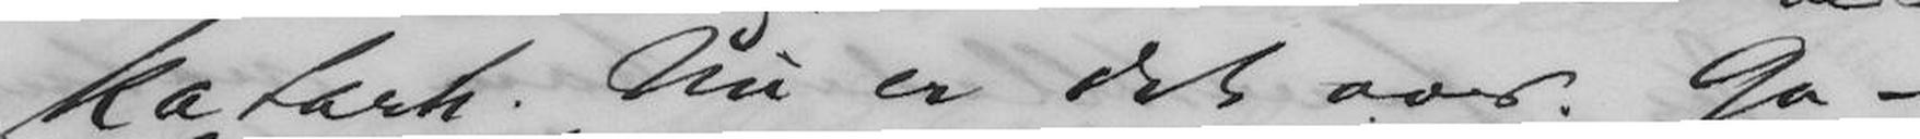katarh. Nu er det over. Ga-
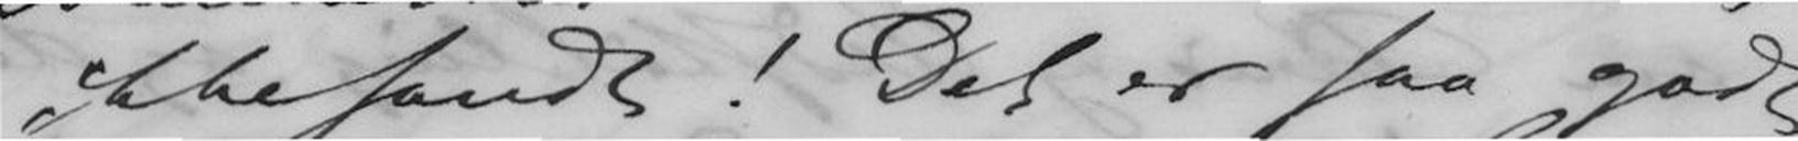ikkesandt! Det er saa godt
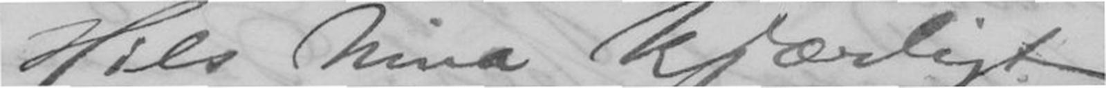Hils Nina kjærligt!
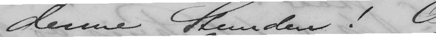denne Stunden! Og
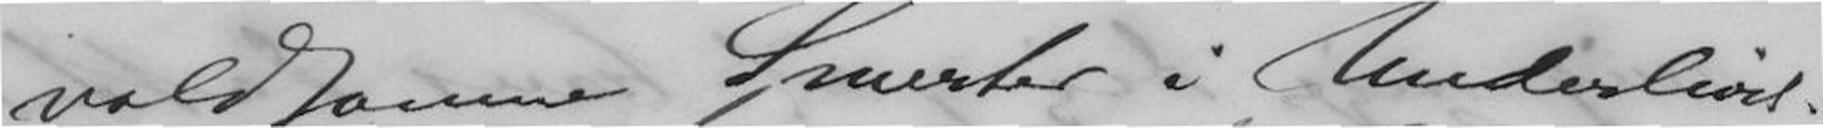voldsomme Smerter i Underlivet.
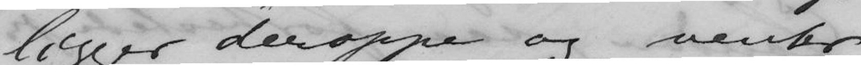ligger deroppe og venter
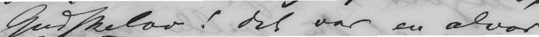Gudskelov! Det var en alvor-
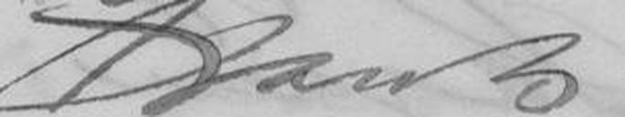Frants.
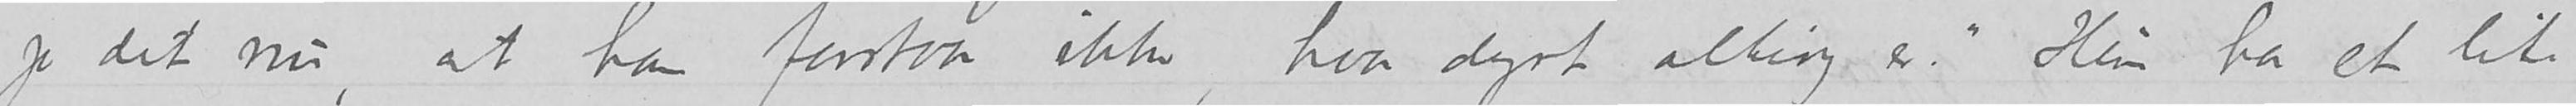jo det nu, at han forstaar ikke, hvor dyrt alting er.»  Hun har et lite
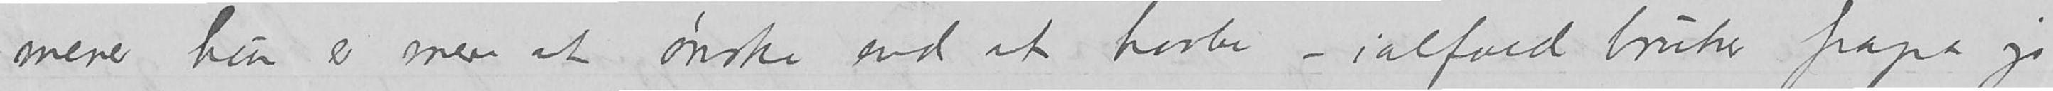mener hun er mere at ønske end at haabe – ialfald bruker papa jo
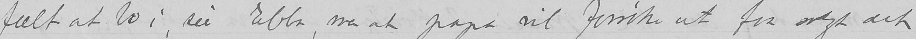fælt at bo i, sier Ebba, men at papa vil forsøke at faa solgt det
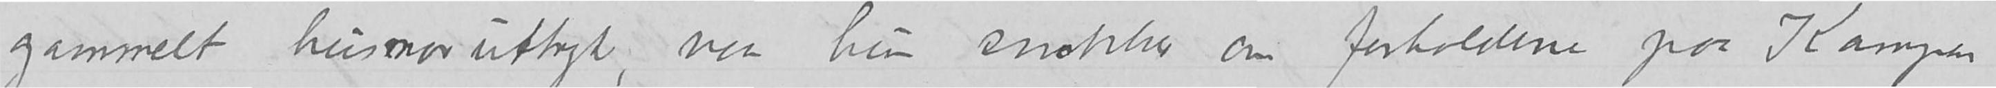gammelt husmoruttryk, naar hun snakker om forholdene paa Kampen
